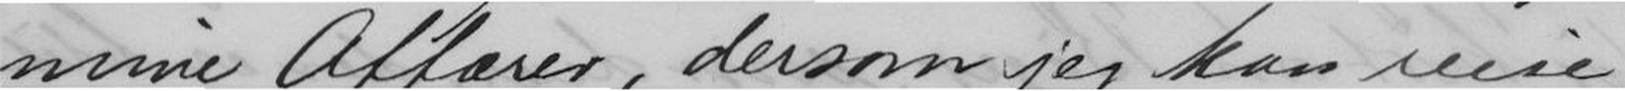mine Affærer, dersom jeg kan vise
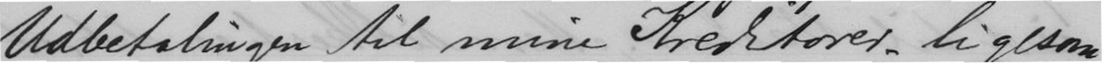Udbetalingen til mine Kreditorer, ligesom
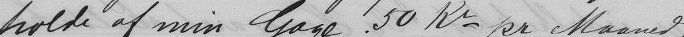holde af min Gage 50 Kr pr Maaned,
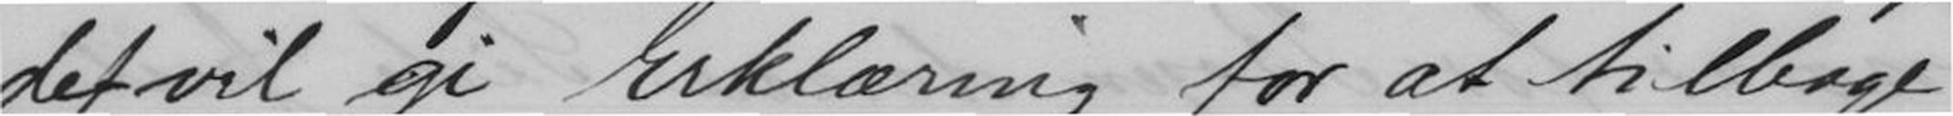det vil gi Erklæring for at tilbage-
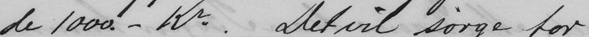de 1000. - Kr. Det vil sørge for
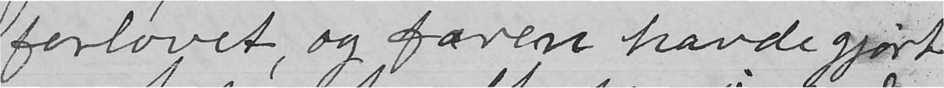forlovet, og faren havde gjort
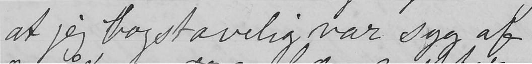at jeg bogstavelig var syg af
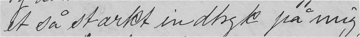et så stærkt indtryk på mig,
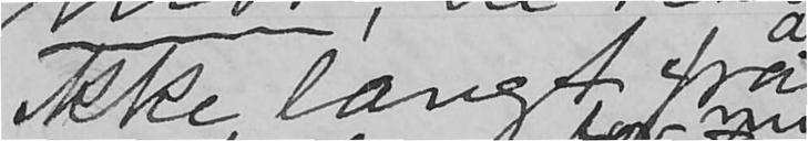ikke langt fra
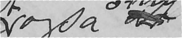også er
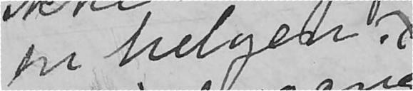en helgen
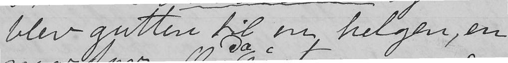blev gutten til en helgen, en
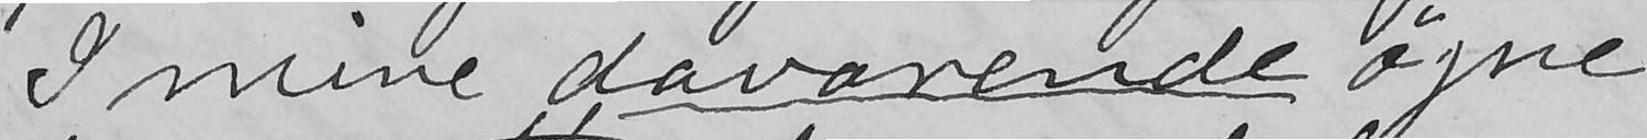I mine daværende øjne
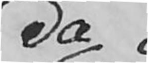da
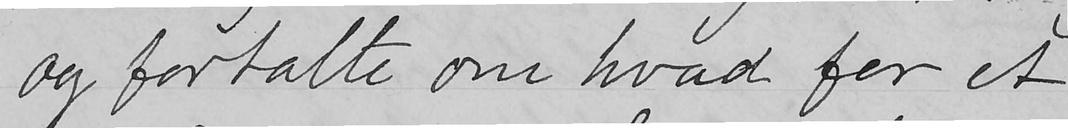og fortalte om hvad for et
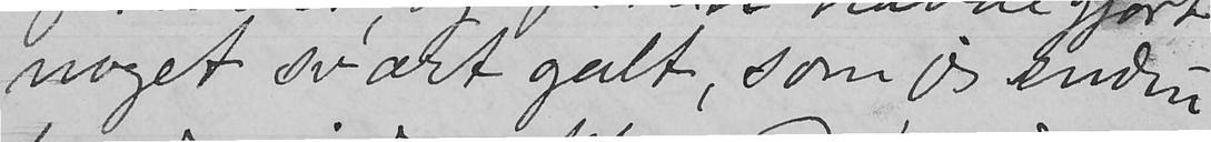noget svært galt, som jeg endnu
